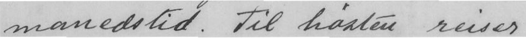månedstid. Til høsten reiser
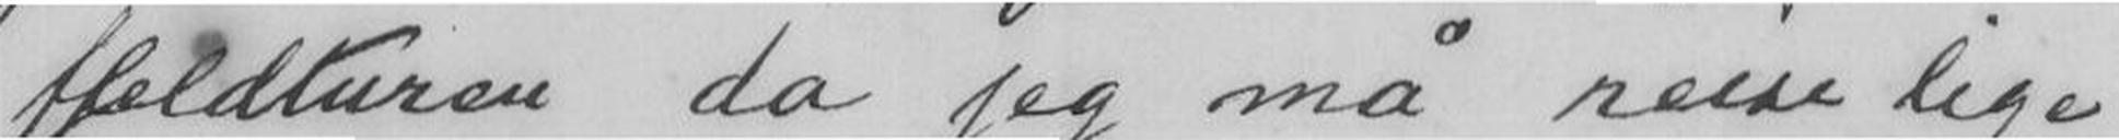fjeldturen da jeg må reise lige
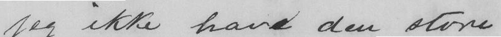jeg ikke have den store
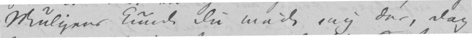Muligens kunde Du møde mig der, dog
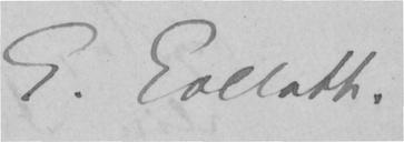C. Collett.
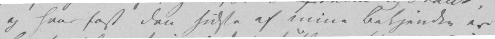og hvor fast den sidste af mine Bekjendte er
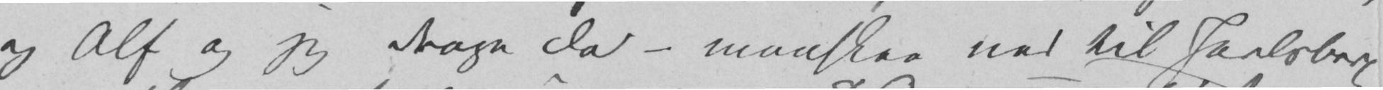og Alf og jeg drage da – maaskee ned til Jarlsberg
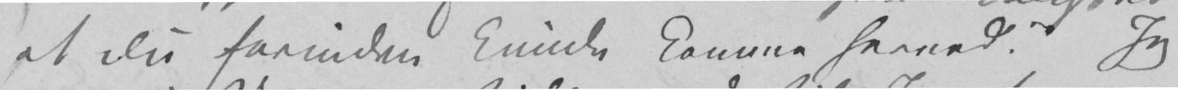at Du forinden kunde komme herned? Jeg
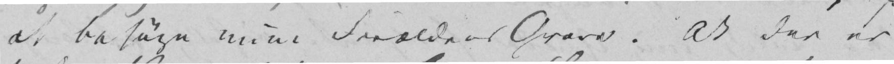at besøge mine Forældres Grave. Ak der er
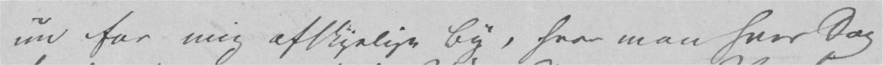nu for mig afskyelige By, hvor man hver Dag
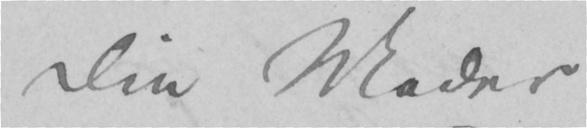Din Moder
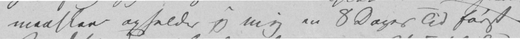maaskee opholder jeg mig en 8 Dages Tid først
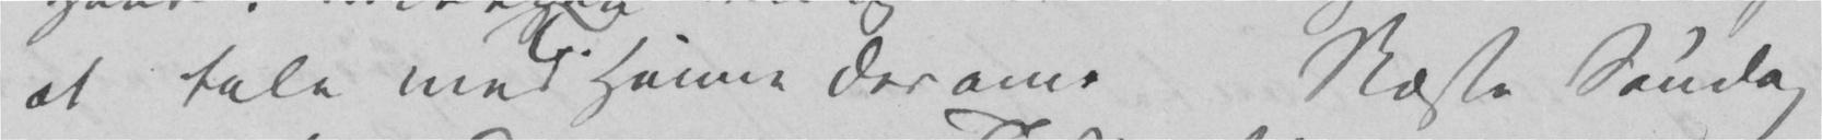at tale med T. Hanne derom. Næste Søndag
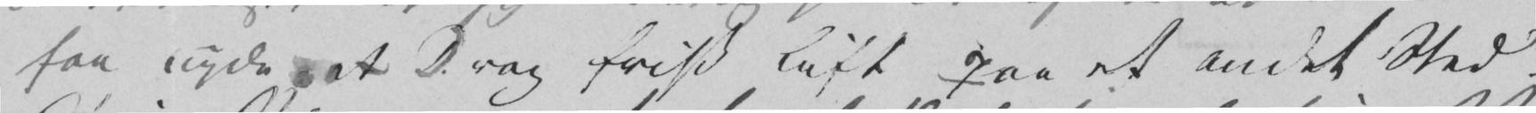faa nyde et Drag frisk Luft paa et andet Sted.
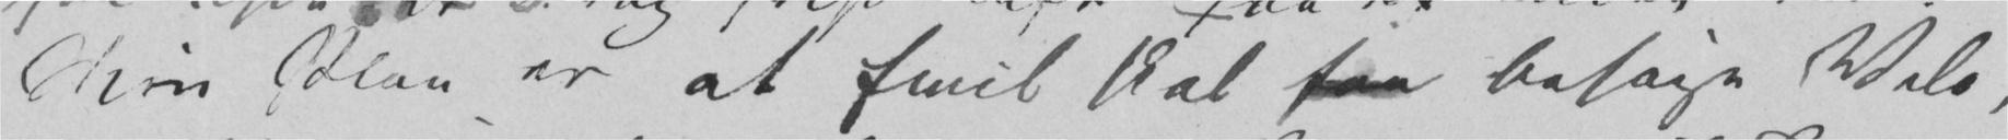Min Plan er at Emil skal faa besøge Velo,
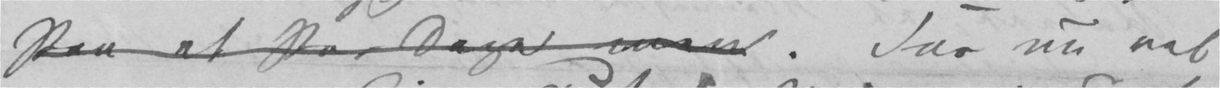Paa et Par Dage men. Far nu vel
